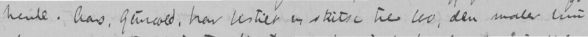hende. Aars, Gunvald, har bestilt en skitse til 100, den maler hun
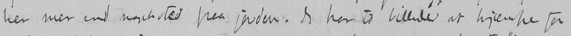her mer end noget sted paa jorden. Jeg har to billeder at kjæmpe for
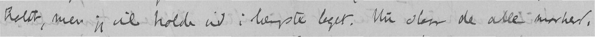koldt, men jeg vil holde ud i længste laget. Nu slaar de alle marker,
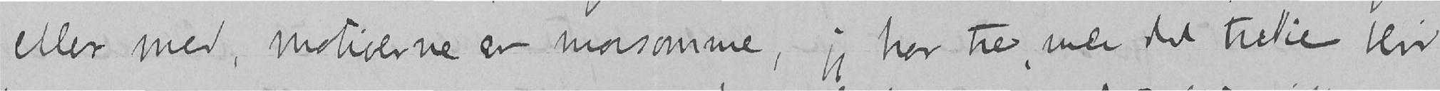eller med, motiverne er morsomme, jeg har tre, men det tredie blir
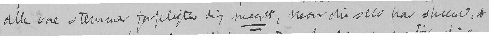alle vore stemmer forpligter dig meget, naar du selv har skrevet, a
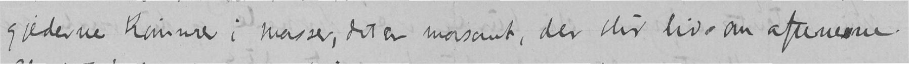gjederne kommer i masser, det er morsomt, der blir liv om aftenerne.
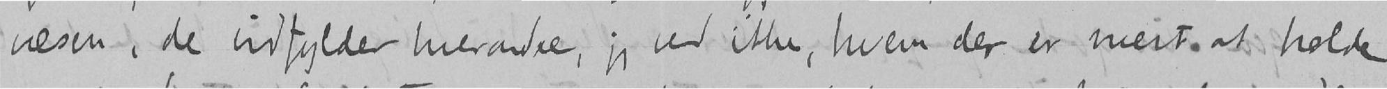væsen, de udfylder hverandre, jeg ved ikke, hvem der er mest at holde
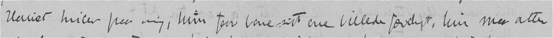Harriet hviler paa mig, hun faar bare sit ene billede færdigt, hun maa atter
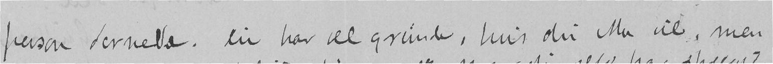person dernede. Du har vel grunde, hvis du ikke vil, men
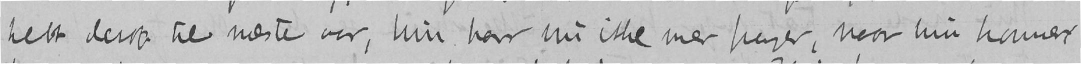helt derop til næste aar, hun har nu ikke mer penger, naar hun kommer
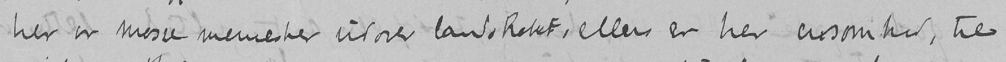her er masse mennesker udover landskabet, ellers er her ensomhed, til
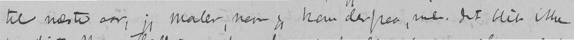til næste aar, jeg maler, naar jeg kan derpaa, men det blir ikke
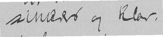sikker og klar.
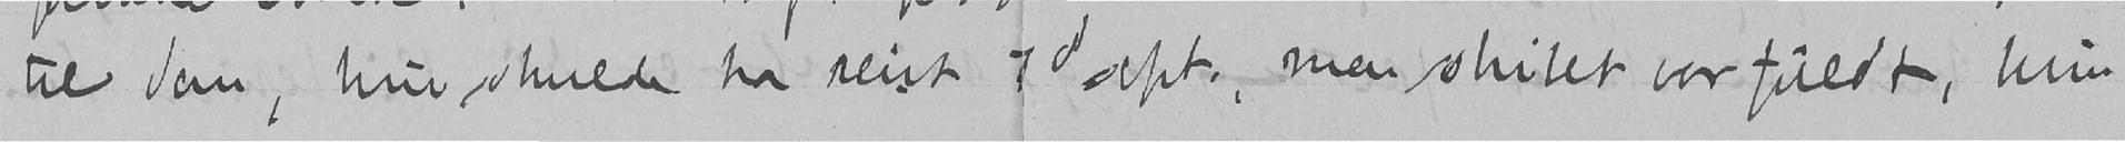til dem, hun skulde har reist 7de sept, men skibet var fuldt, hun
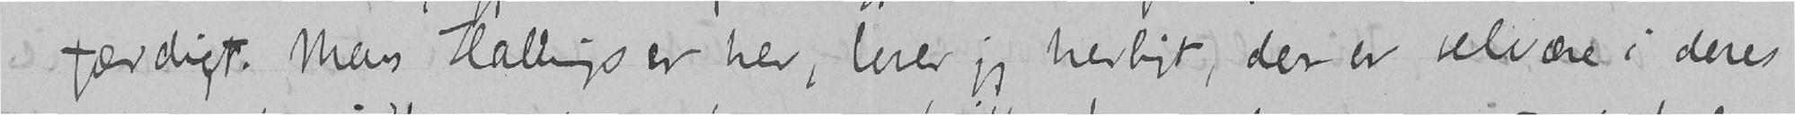færdigt. Mens Hallings er her, lever jeg herligt, der er velvære i deres
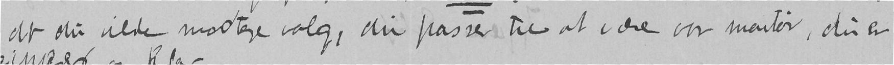at du vilde modtage valg, du passer til at være vor montør, du er
